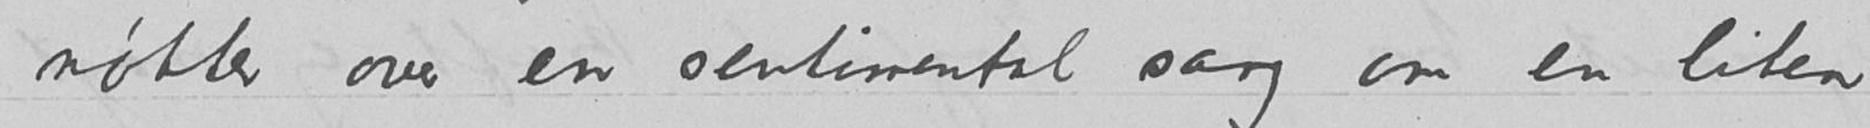nøtter over en sentimental sang om en liten
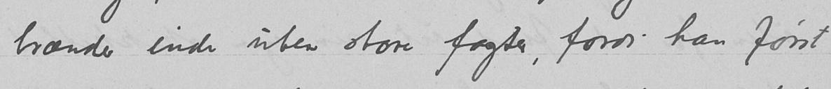brænder inde uten store fagter, fordi han først
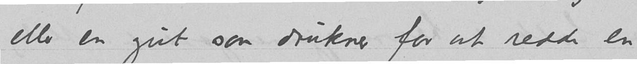eller en gut som drukner for at redde en
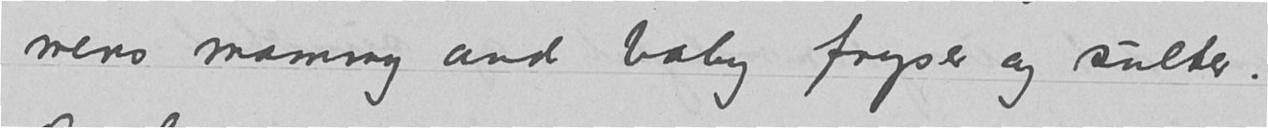mens manmy and baby fryser og sulter.
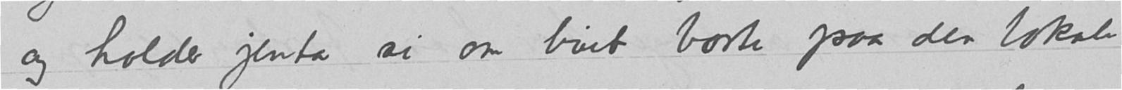og holder jenta si om livet borte paa den lokale
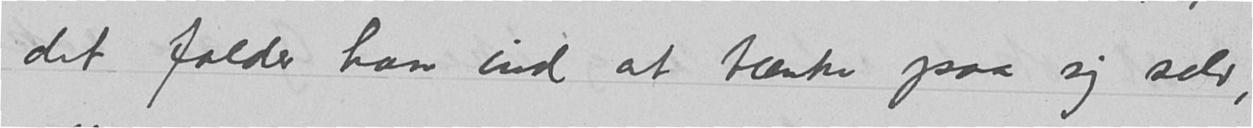det falder ham ind at tænke paa sig selv,
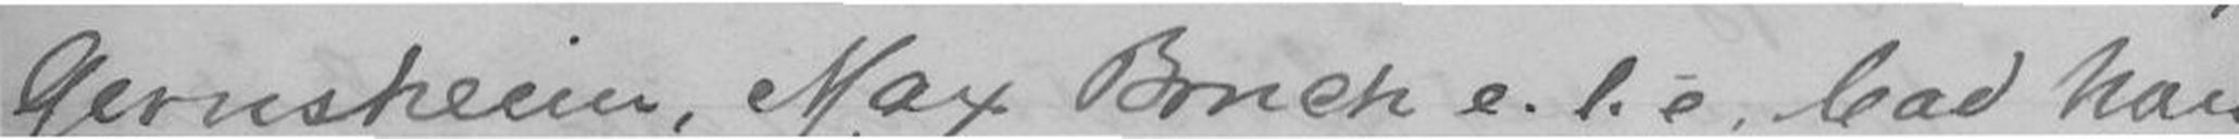Gernsheim, Max Borch e.l.e. bad han
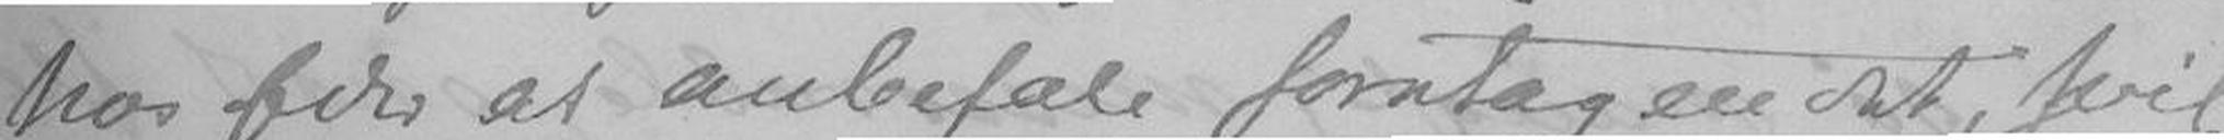hos Eder at anbefale foretagendet, hvil-
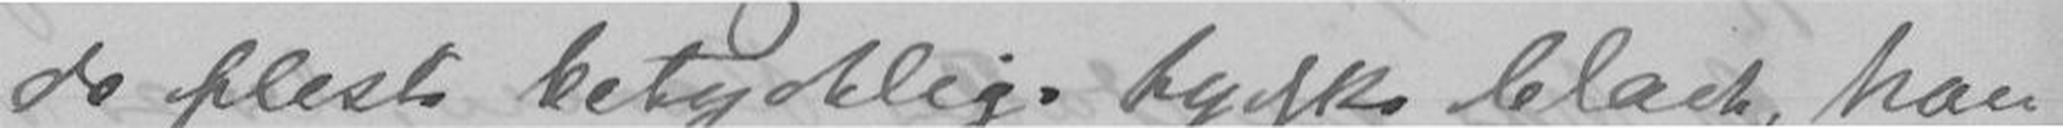de fleste betydelige tydske blad, han
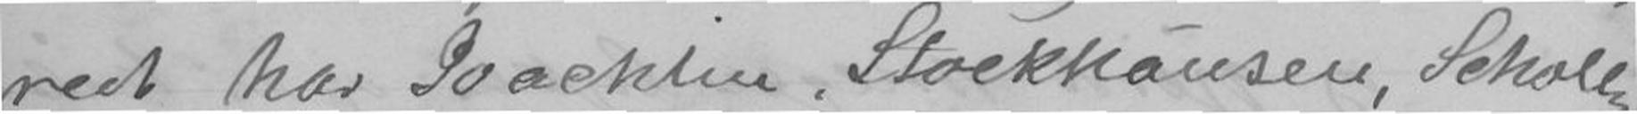rede har Joackim, Stockhausen, Scholtz
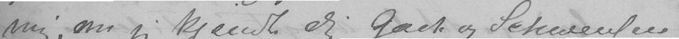mig, om jeg kjendte dig Gach og Schwenlm
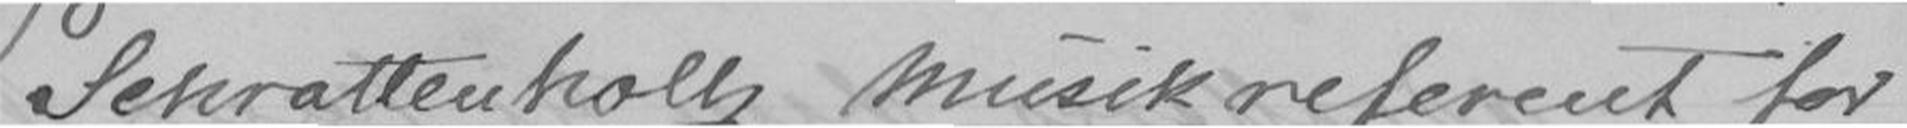Schrattenholtz Musikreferent for
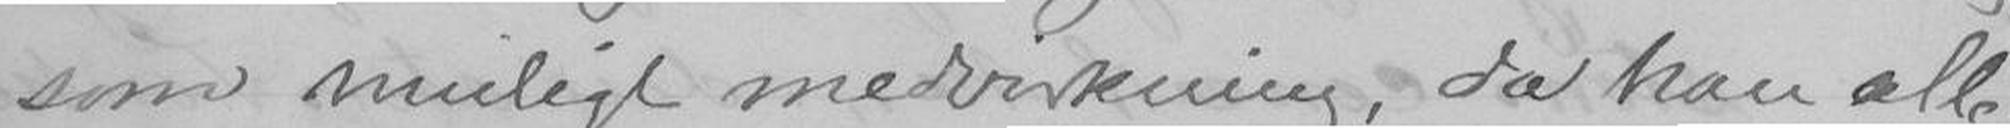som muligt medvirkning, da han alle-
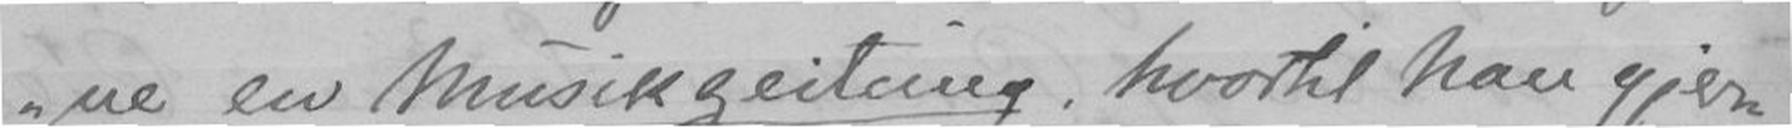ve en Musikzeitung, hvortil han gjerne
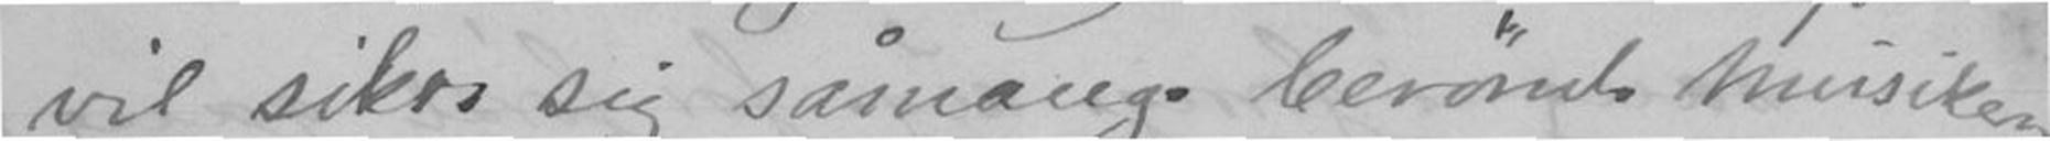vil sikre sig såmange berømde Musikere
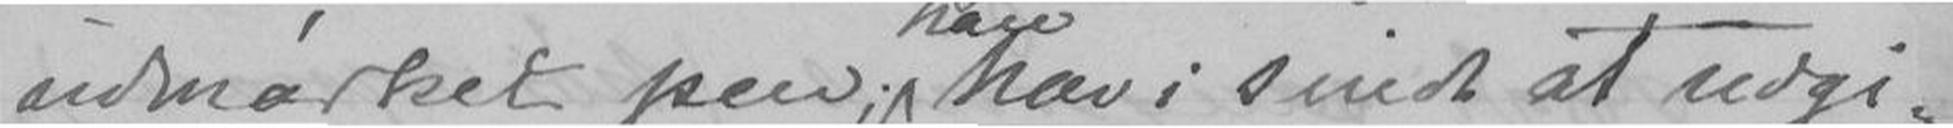udmærket pen; han har i sindt at udgi-
